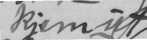kjem ut
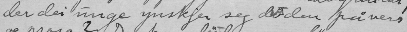der dei unge ynskjer seg døden på vers
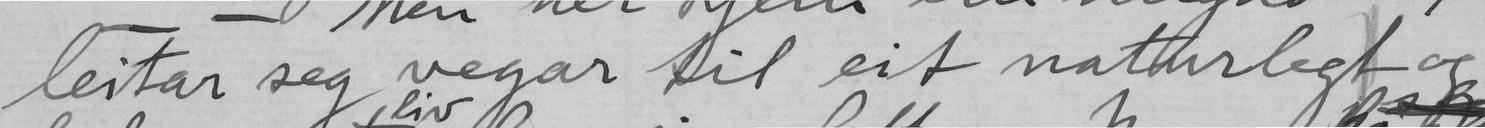leitar seg vegar til eit naturlegt og
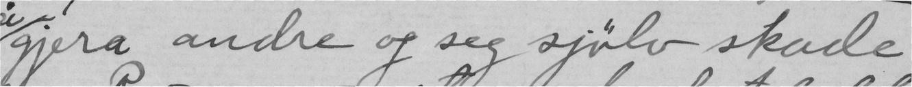gjera andre og seg sjølv skade
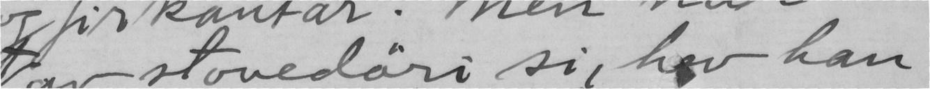av stovedøri si, hev han
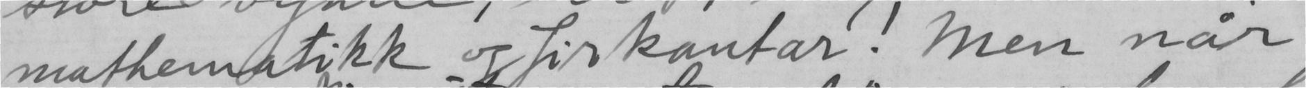mathematikk og firkantar! Men når
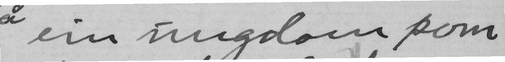ein ungdom som
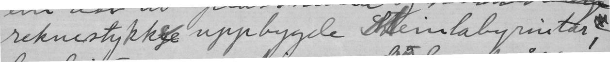reknestykkje uppbygde steinlabyrintar,
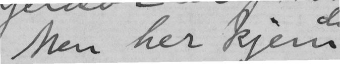- - Men her kjem
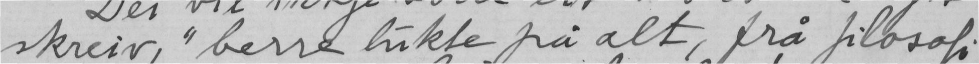skreiv, "berre lukte på alt, frå filosofi
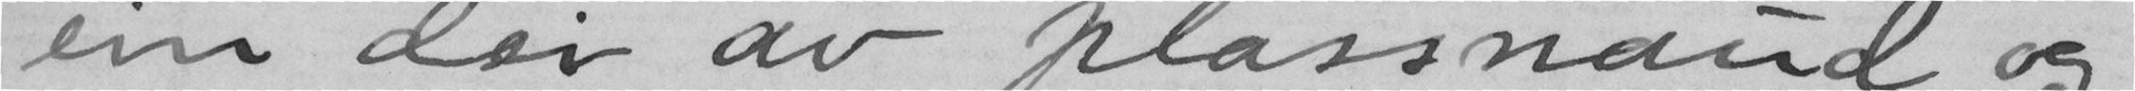ein dei av plassnaud og
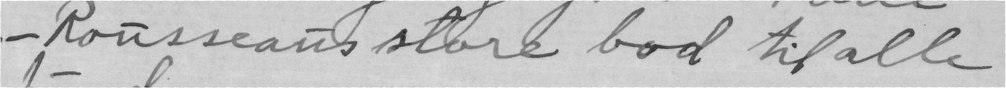- Rousseaus store bod til alle
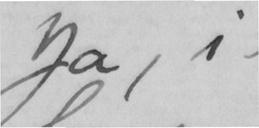Ja, i
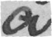å
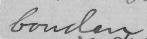bonden
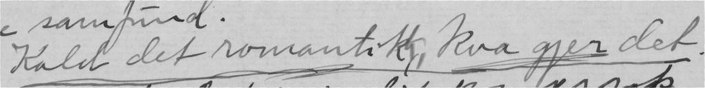Kald det romantik, kva gjer det!
